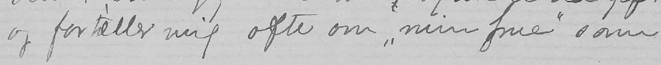og fortæller mig ofte om «min frue» som
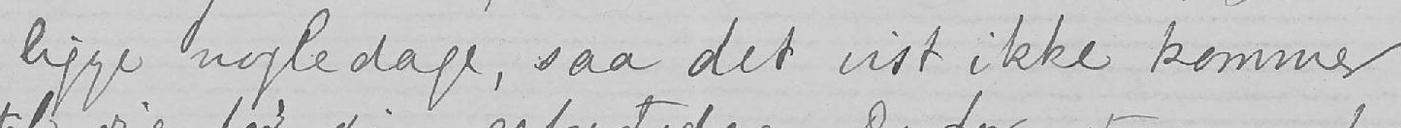ligge nogle dage, saa det vist ikke kommer
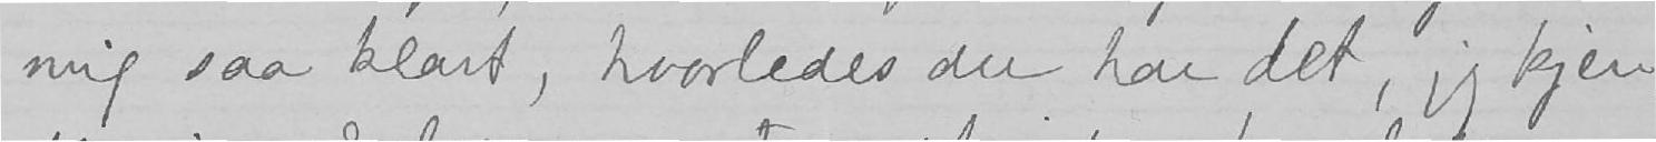mig saa klart, hvorledes du har det, jeg kjen-
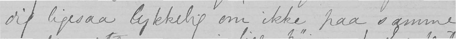dig ligesaa lykkelig om ikke paa samme
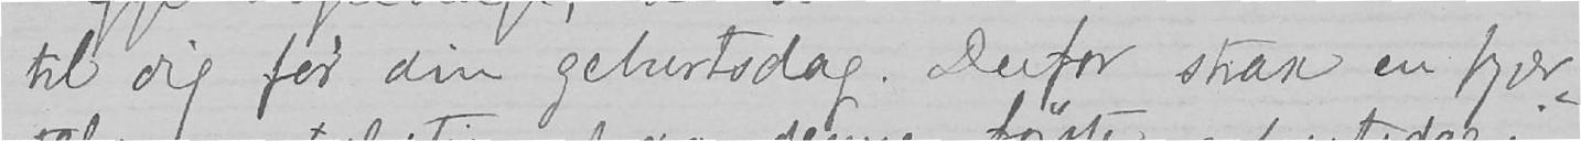til dig før din geburtsdag. Derfor strax en hjær-
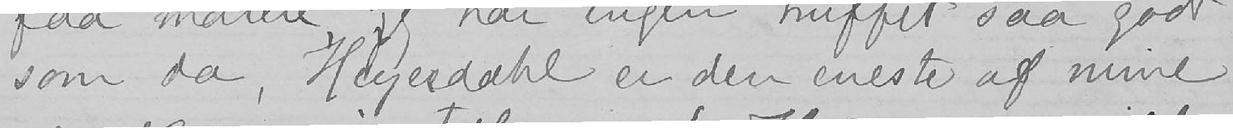som da, Heyerdahl er den eneste af mine
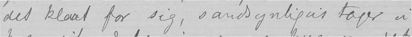det klart for sig, sandsynligvis tager vi
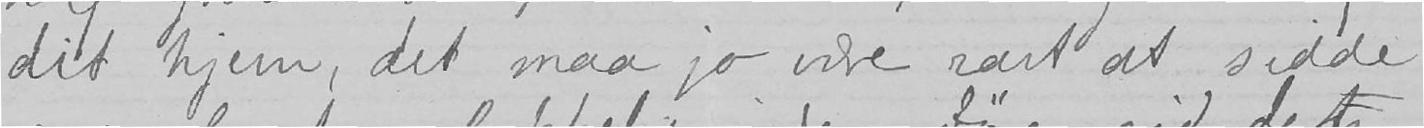dit hjem, det maa jo være rart at sidde
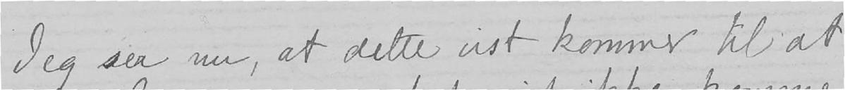Jeg ser nu, at dette vist kommer til at
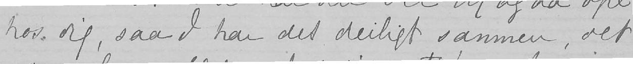hos dig, saa I har det deiligt sammen, det
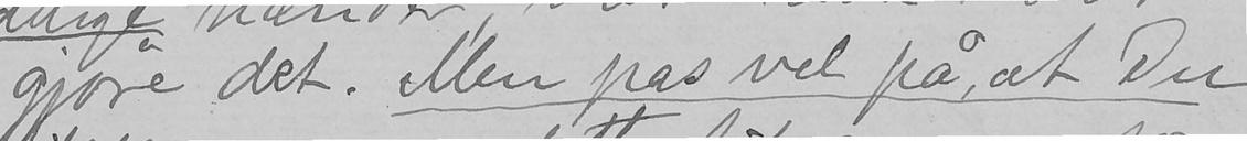gjøre det. Men pas vel på, at Du
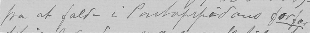fra at falde i Pontoppidans forfær
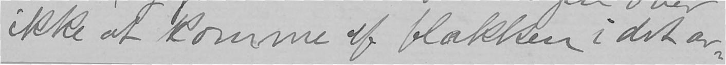ikke at komme af flækken i det ar-
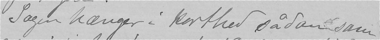Sagen hænger i korthed sådan sam-
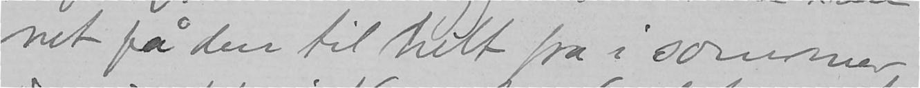net få den til helt fra i sommer
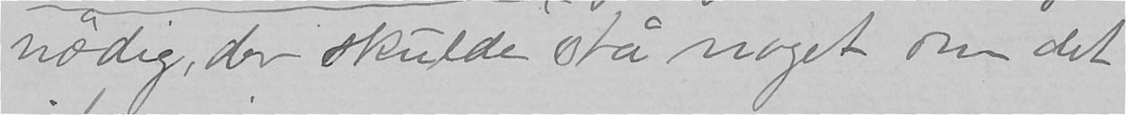nødig, der skulde stå noget om det
